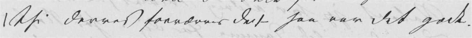(thi derved forværres de) – saa var det godt.
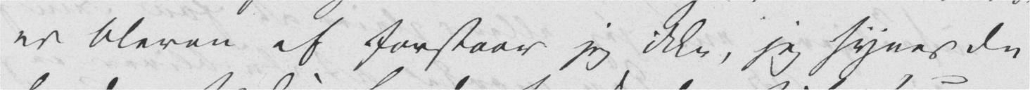er bleven af forstaar jeg ikke, jeg synes Du
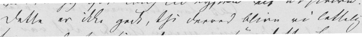Dette er ikke godt, thi derved blive vi lettelig
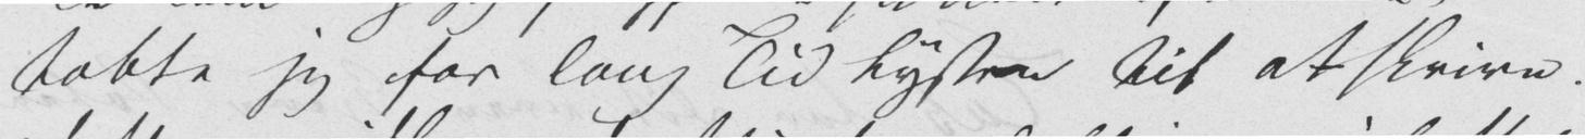tabte jeg for lang Tid Lysten til at skrive.
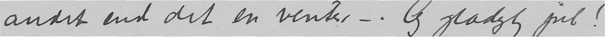andet end det en venter , Og glædelig jul!
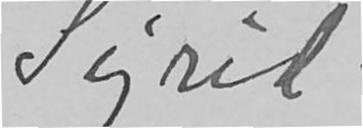Sigrid.
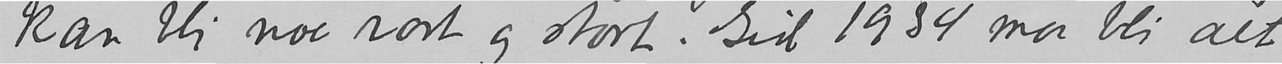kan bli noe rart og stort. Gid 1934 maa bli alt
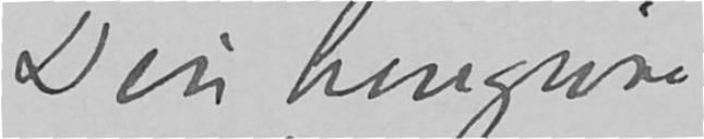Din hengivne
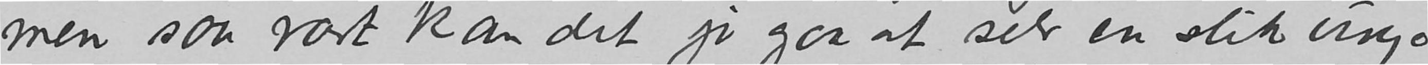men saa rart kan det jo gaa at selv en slik unge
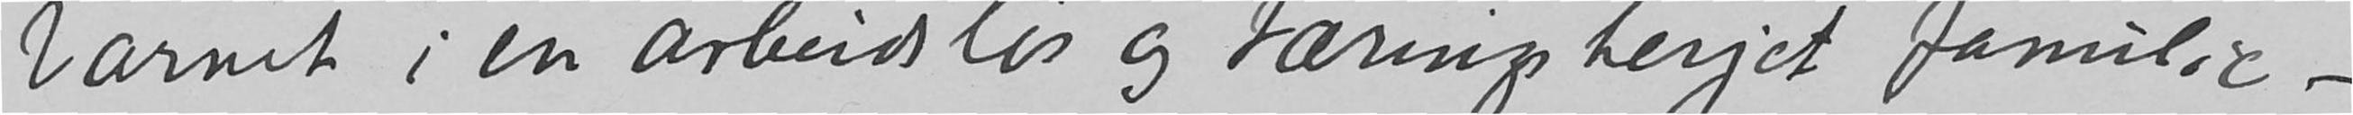barnet i en arbeidsløs og tæringsherjet familie,
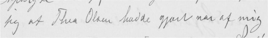jeg at Thea Olsen hadde gjort nar af mig
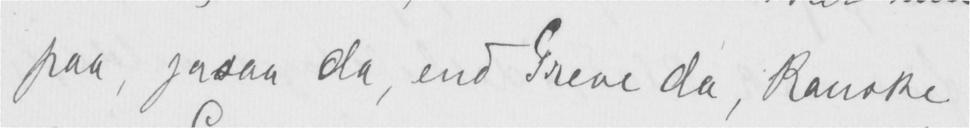paa, jasaa da, end Greve da, kanske
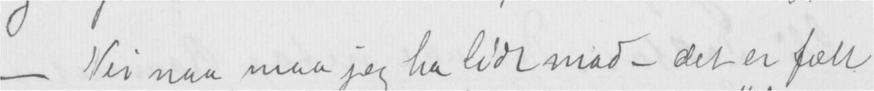– Nei naa maa jeg ha lidt mad – det er fælt
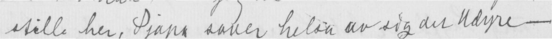stille her, Sjapa sover helsa av sig det udyre –
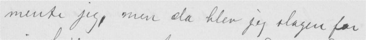mente jeg, men da blev jeg slagen for
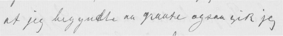at jeg begyndte aa graate ogsaa gik jeg
